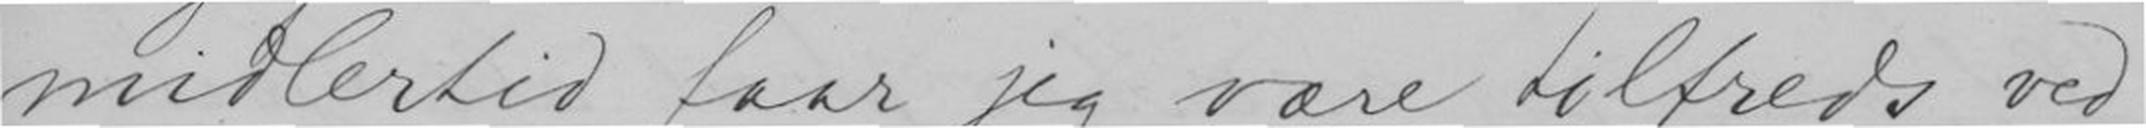midlertid faar jeg være tilfreds ved
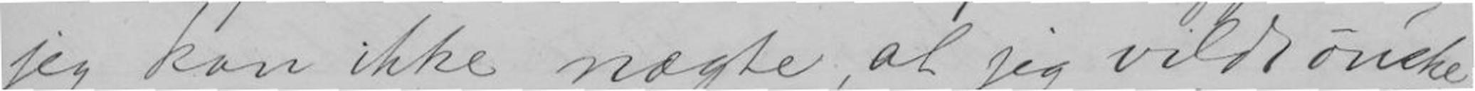jeg kan ikke nægte, at jeg vilde ønske,
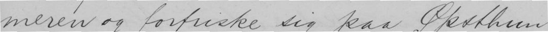meren og forfriske sig paa Øpsthun.
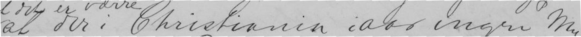at det er værre at der i Christiania iaar ingen Mu-
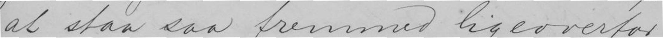at staa saa fremmed ligeoverfor
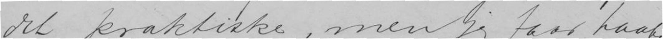det praktiske, men jeg faar haabe
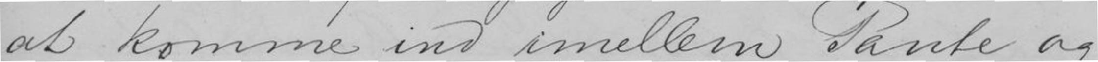at komme ind imellem Pante og
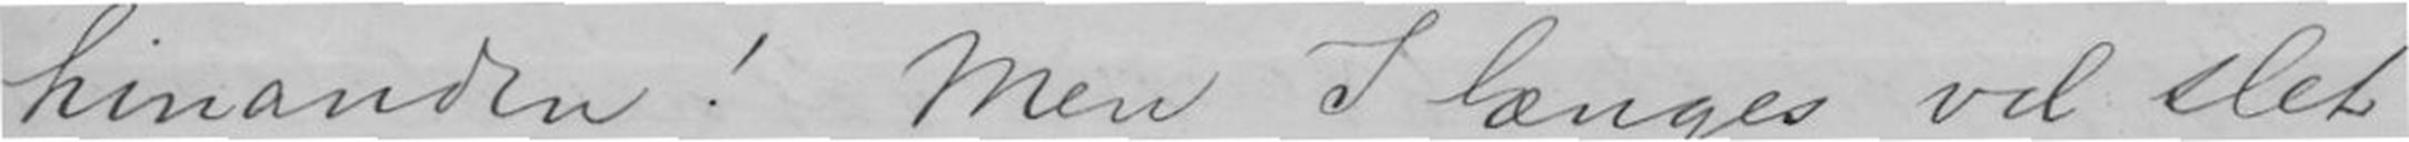hinanden! Men I længes vel slet
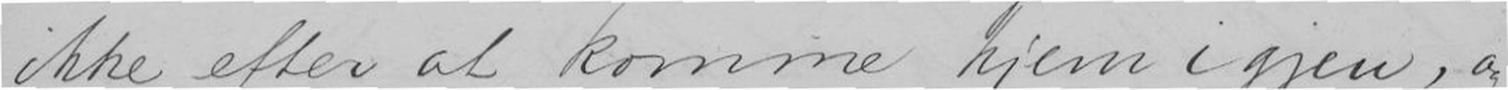ikke efter at komme hjem igjen, og
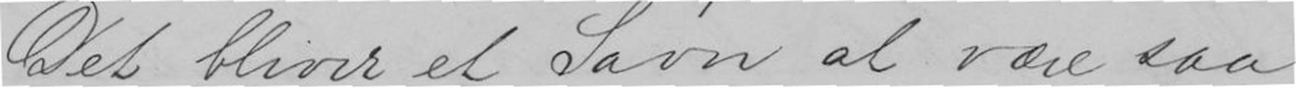Det bliver et Savn at være saa
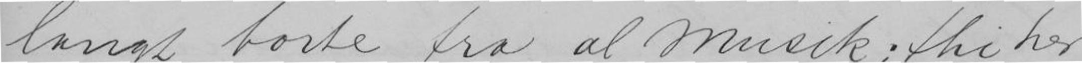langt borte fra al Musik; thi her
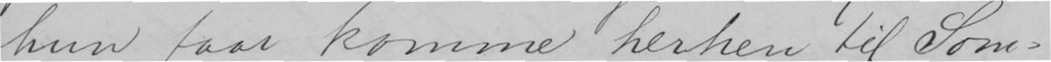hun faar komme herhen til Som-
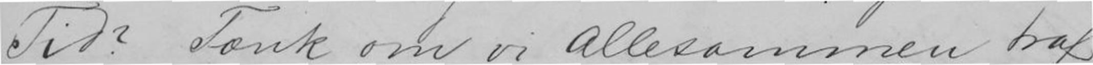Tid? Tænk om vi Allesammen traf
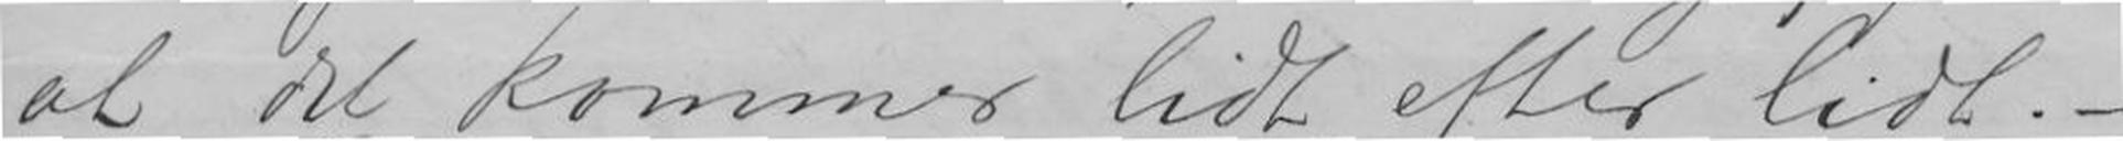at det kommer lidt efter lidt. -
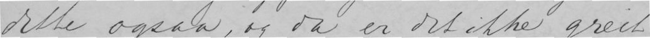dette ogsaa, og da er det ikke greit
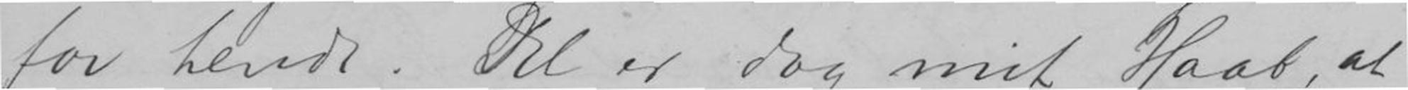for hende. Det er dog mit Haab, at
